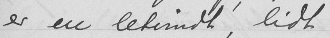er en letvindt, lidt
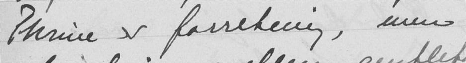Thrine er forretning, men
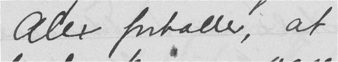Alex fortæller, at
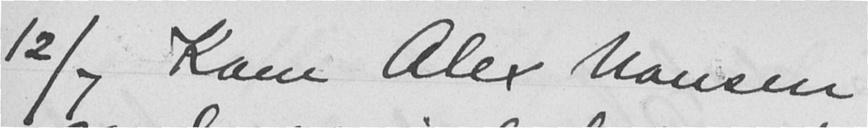12/7 Kom Alex Nansen
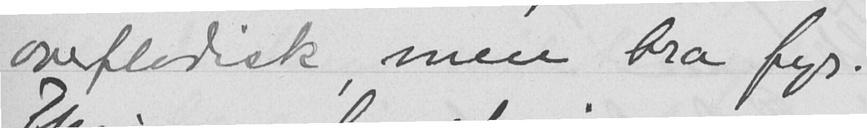overfladisk, men bra fyr.
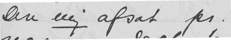Dere mig afsat pr.
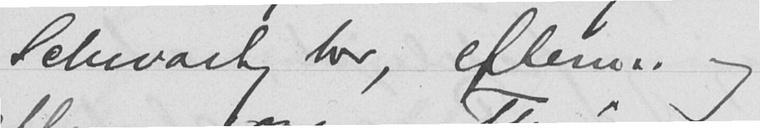Schwartz her, efterm. og
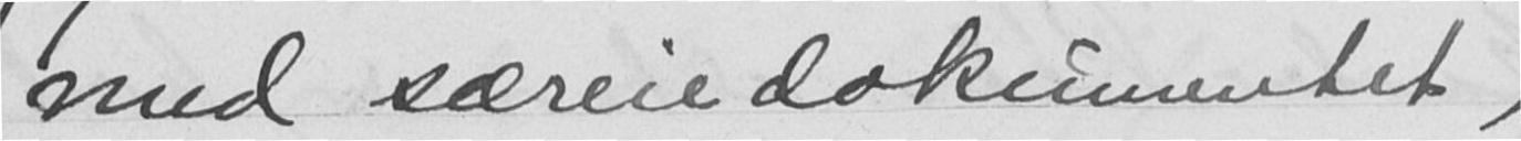med særeiedokumentet,
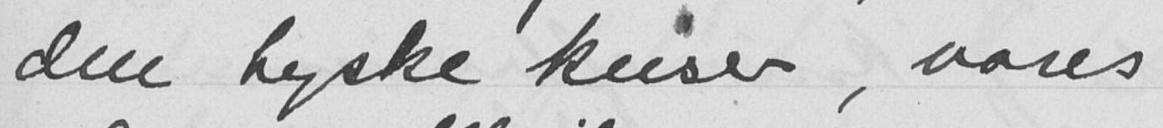den tyske keiser, vores
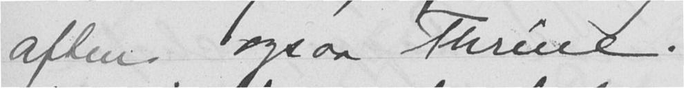aften ogsaa Thrine.
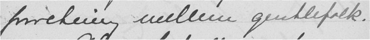forretning mellem gentlefolk.
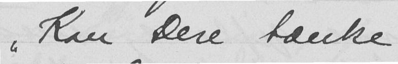"Kan Dere tænke
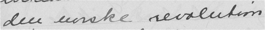den norske revolution
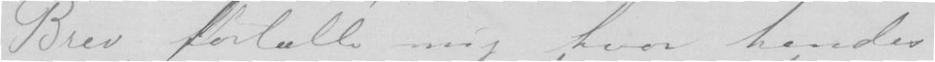Brev fortalde mig hvor hendes
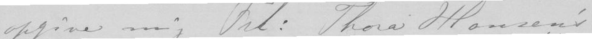opgive mig Frk. Thora Hansens
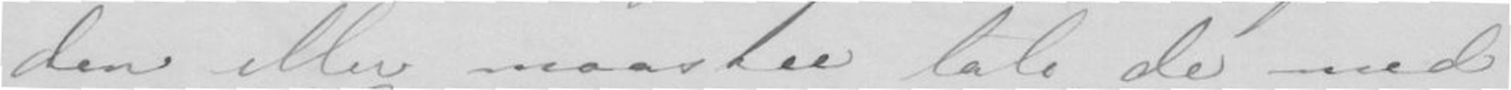den eller maaskee tale de med
